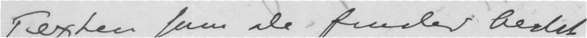Texten som De finder bedst
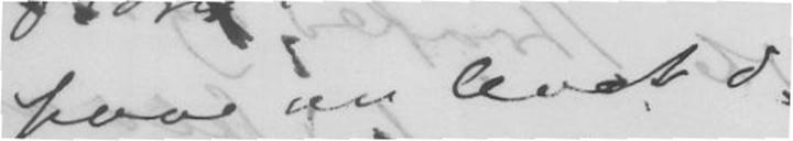saa nu livet i
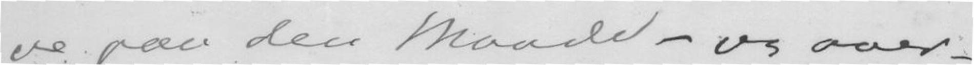ve paa den Maade - vi over-
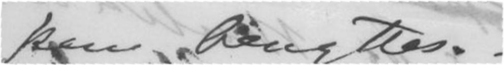kan Benyttes. -
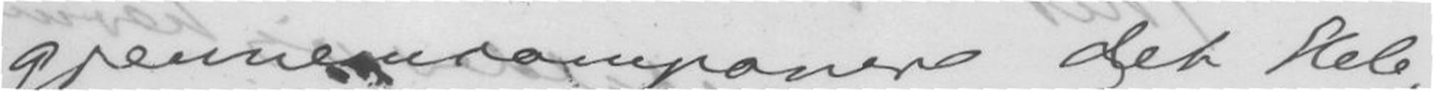gjennemcomponere det Hele,
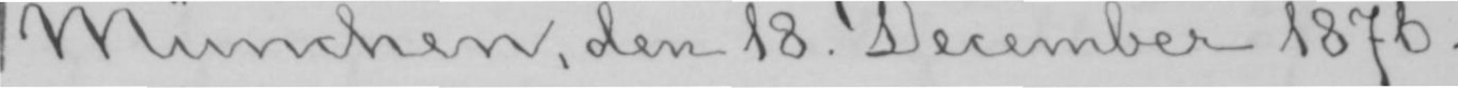München, den 18. December 1876.
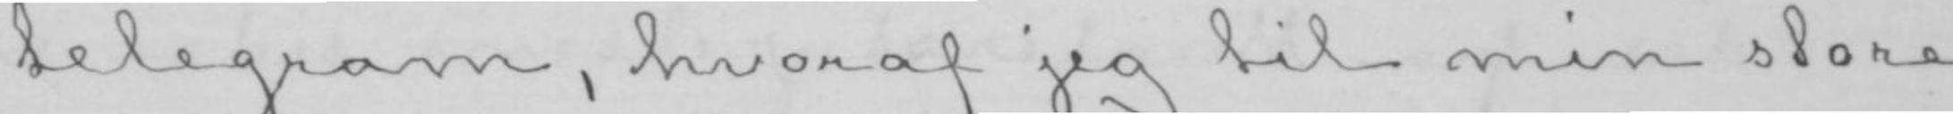telegram, hvoraf jeg til min store
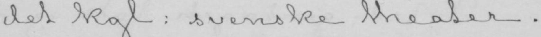det kgl: svenske theater.
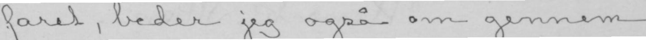faret, beder jeg også om gennem
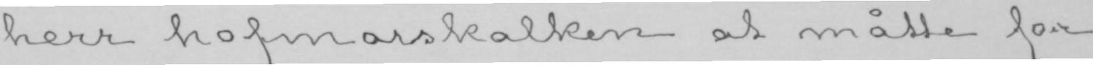herr hofmarskalken at måtte for
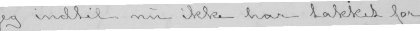jeg indtil nu ikke har takket for
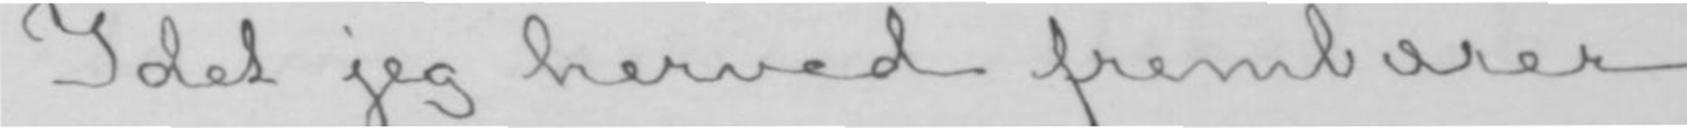Idet jeg herved frembærer
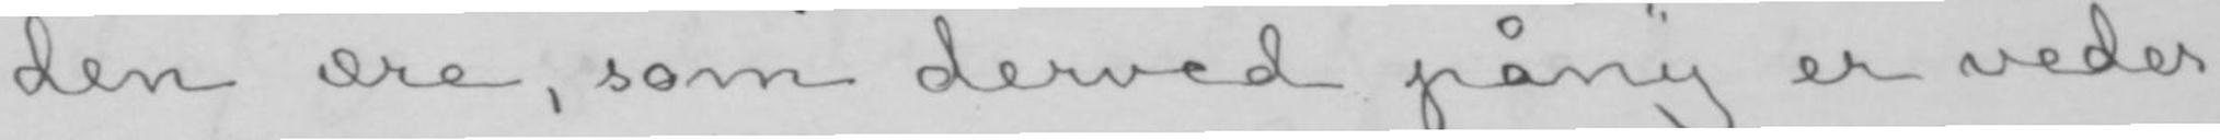den ære, som derved påny er veder-
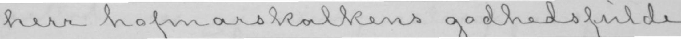herr hofmarskalkens godhedsfulde
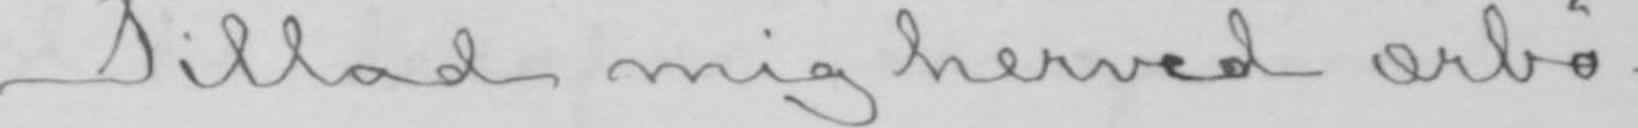Tillad mig herved ærbø-
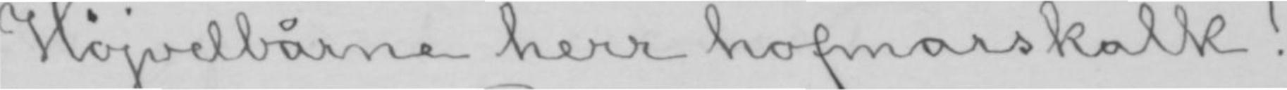Højvelbårne herr hofmarskalk!
